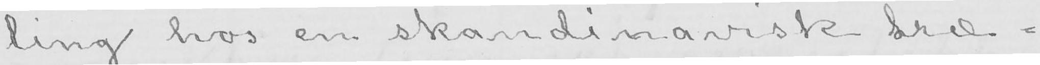ling hos en skandinavisk træ-
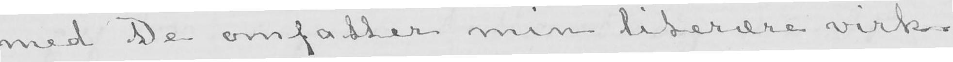med De omfatter min literære virk-
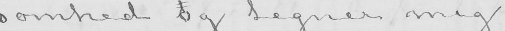somhed tog tegner mig
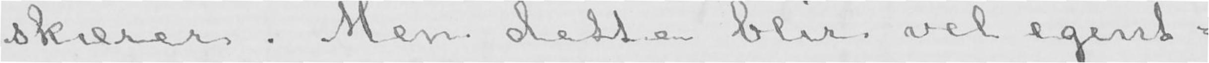skærer. Men dette blir vel egent-
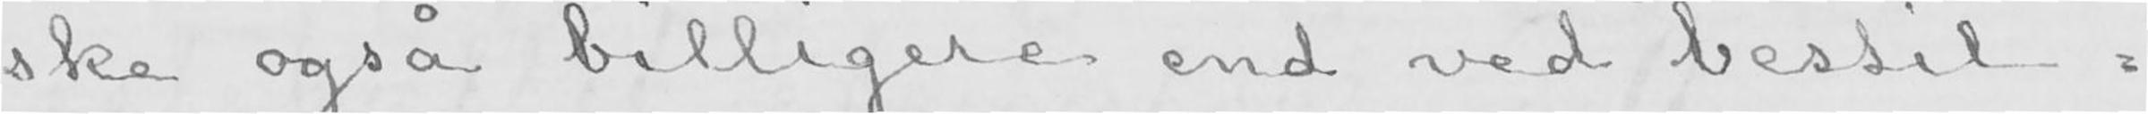ske også billigere end ved bestil-
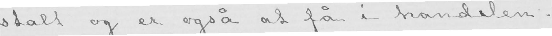stalt og er også at få i handelen.
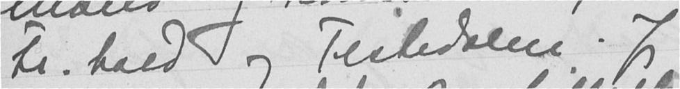Fr. hald og Tistedalen. Jeg
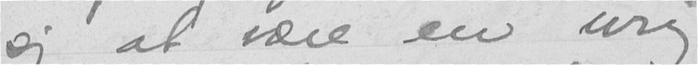sig at være en ivrig
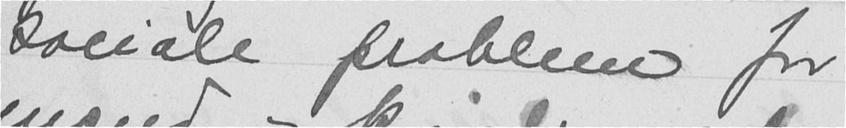sociale problem for
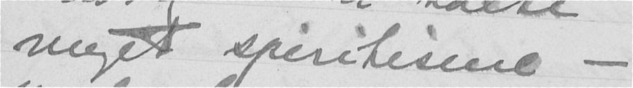meget spiritisme -
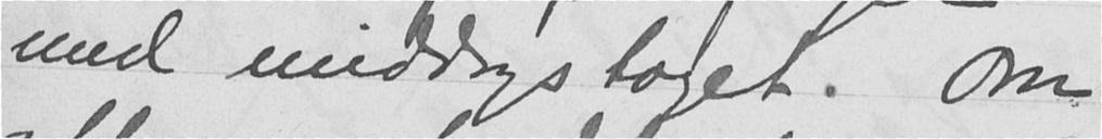med middagstoget. Om
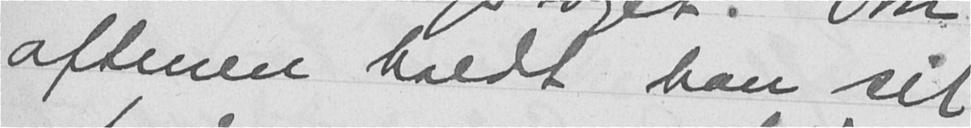aftenen holdt han sit
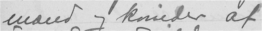mænd og kvinder af
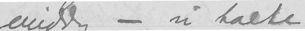middag - vi talte
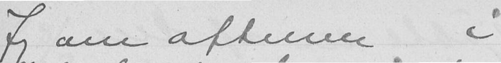Jeg om aftenen i
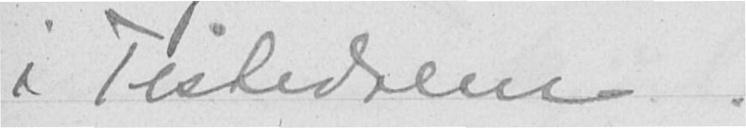i Tistedalen.
